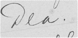Dea.
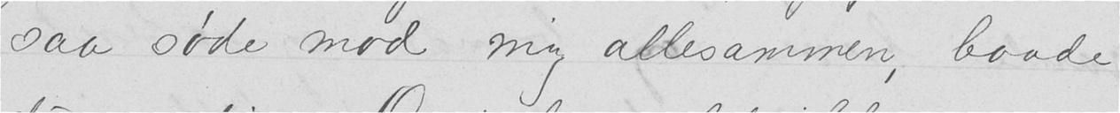saa søde mod mig allesammen, baade
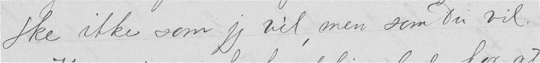Ske ikke som jeg vil, men som Du vil.
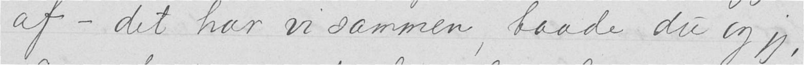af - det har vi sammen,  baade du og jeg,
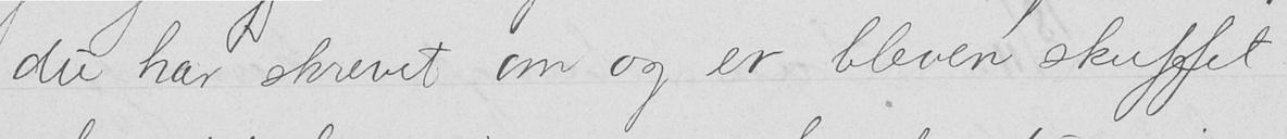du har skrevet om og er bleven skuffet
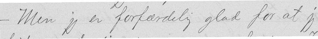- Men jeg er forfærdelig glad for at jeg
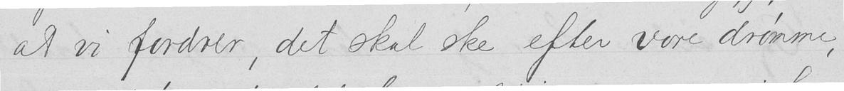at vi fordrer, det skal ske efter vore drømme,
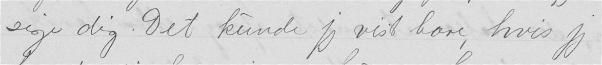sige dig. Det kunde jeg vist bare, hvis jeg
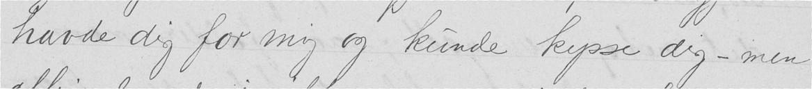havde dig for mig og kunde kysse dig - men
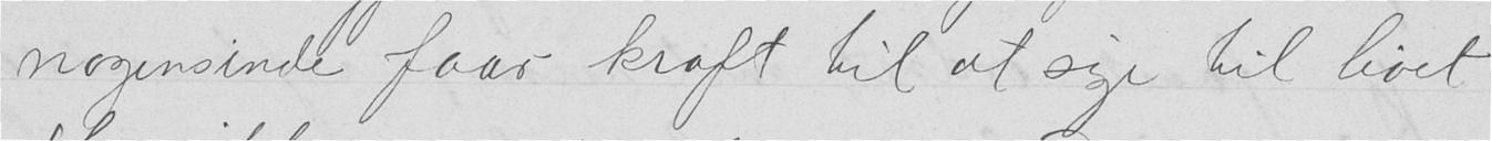nogensinde faar kraft til at sige til livet
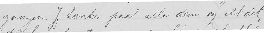gangen. Jeg tænker paa alle dem og alt det,
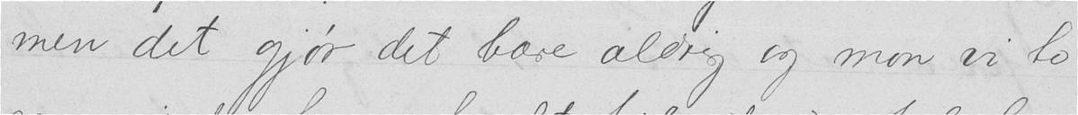men det gjør det bare aldrig og mon vi to
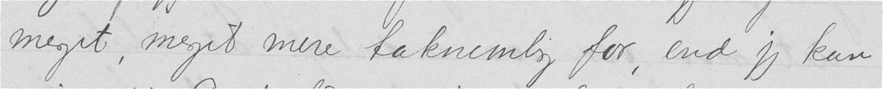meget, meget mere taknemlig for, end jeg kan
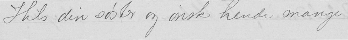Hils din søster og ønsk hende mange
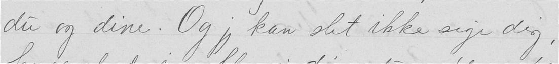du og dine. Og jeg kan slet ikke sige dig,
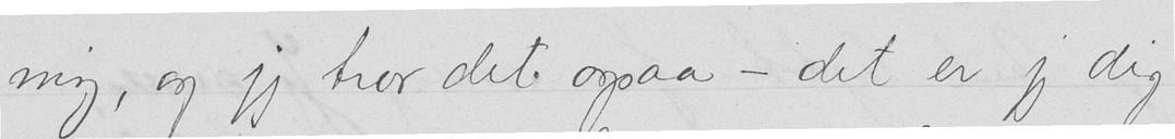mig, og jeg tror det ogsaa - det er jeg dig
